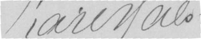Karl Hals.
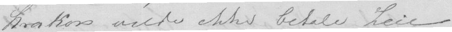Strakors vilde ikke betale Leie
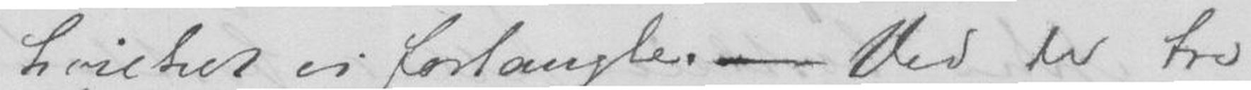hvilket vi forlangte. - Ved de tre
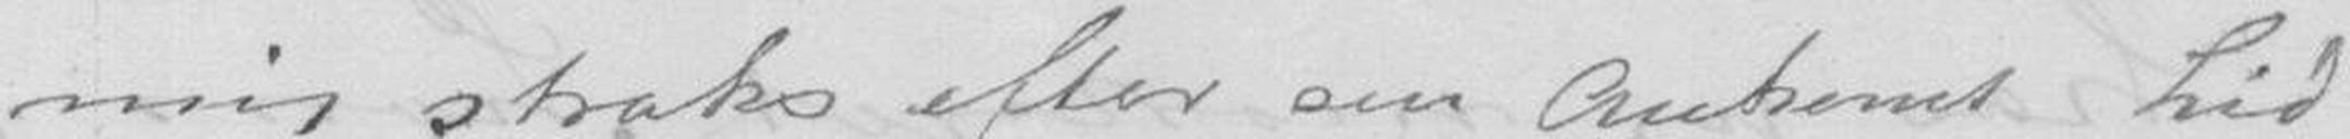mig straks efter sin Ankomst hid
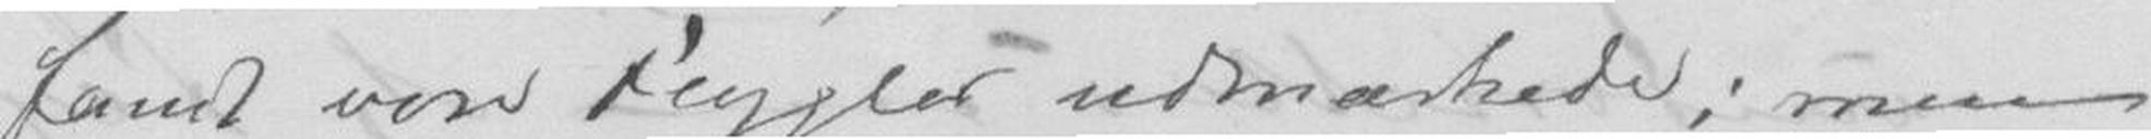fandt vore Flygler udmærkede; men
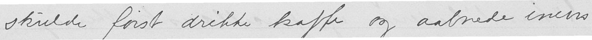skulde først drikke kaffe og aabnede imens
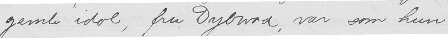gamle idol, fru Dybwad, var som hun
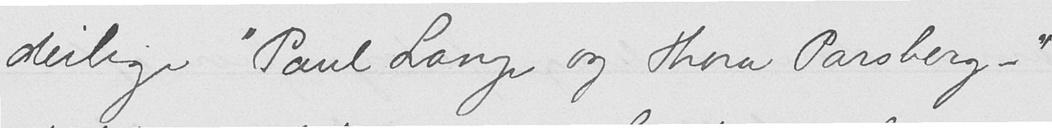deilige "Paul Lange og Thora Parsberg - "
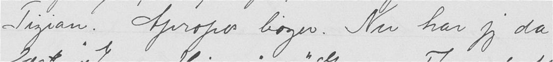Tizian. Apropos bøger. Nu har jeg da
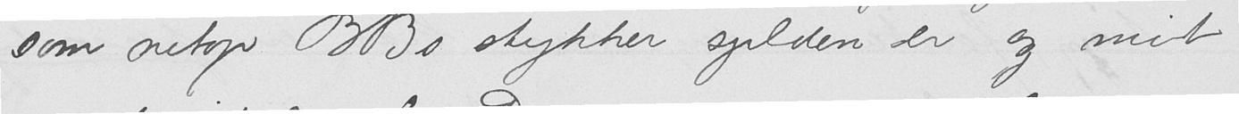som netop BBs stykker sjelden er og mit
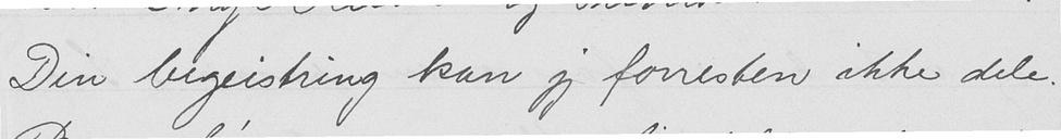Din begeistring kan jeg forresten ikke dele.
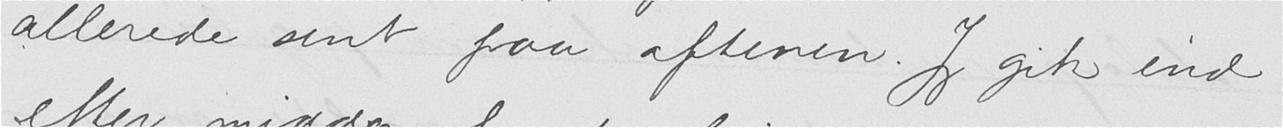allerede sent paa aftenen. Jeg gik ind
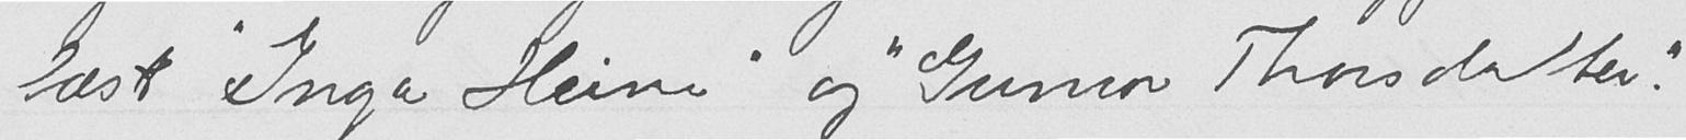læst "Inga Heine" og "Gunvor Thorsdatter".
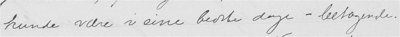kunde være i sine bedste dage - betagende.
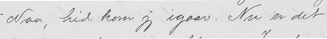- Naa, hid kom jeg igaar. Nu er det
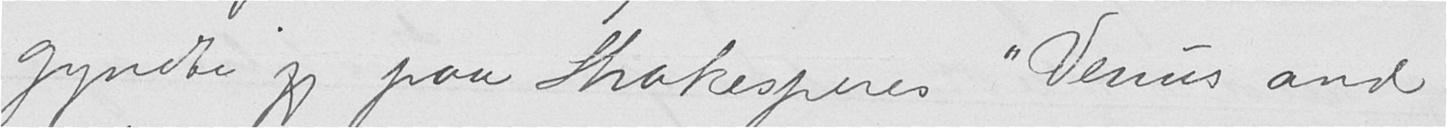gyndte jeg paa Shakespeares "Venus and
